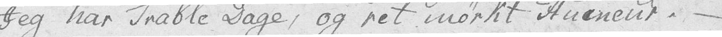Jeg har Trable Dage, og ret mørkt Humeur. –
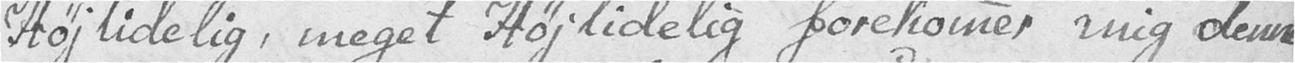Højtidelig, meget Højtidelig forekommer mig denne
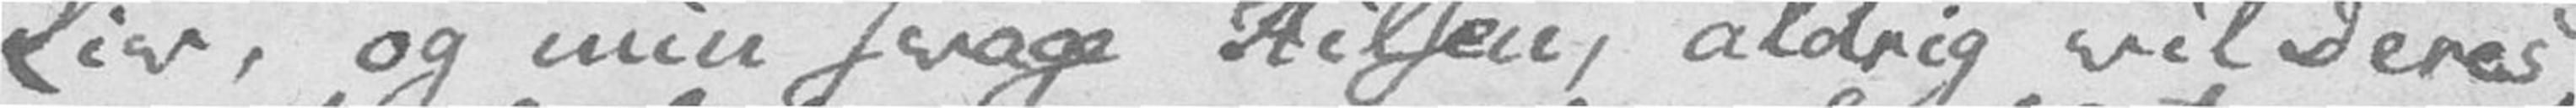Liv, og min svage Hilsen, aldrig vil Deres
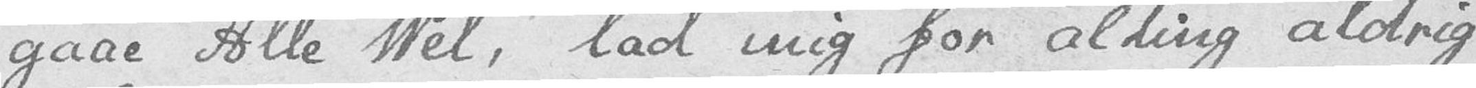gaae Alle Vel, lad mig for alting aldrig
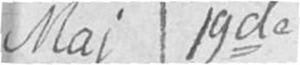Maj 19de
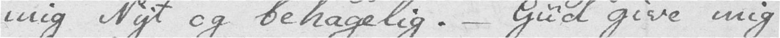mig Nyt og behagelig. – Gud give mig
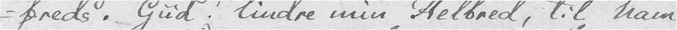-freds. Gud! lindre min Helbred, til ham
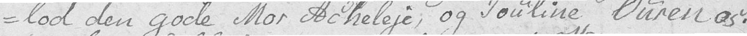-lod den gode Mor Acheleje, og Pouline Ouren os.
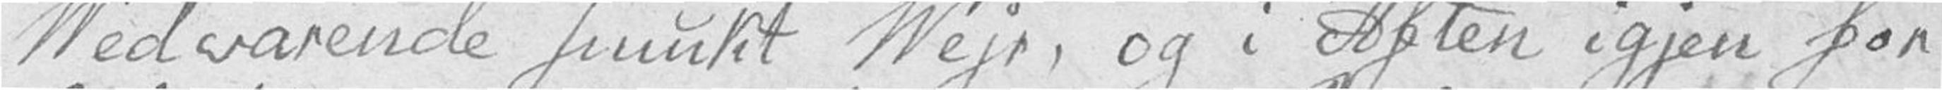Vedvarende smukt Vejr, og i Aften igjen for
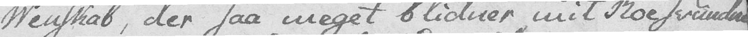Venskab, der saa meget blidner mit Roe svundne
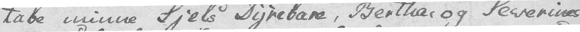tabe minne Sjels Dyrebare, Berthas og Severines
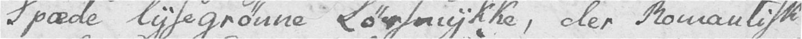Spæde lysegrønne Løvsmykke, der Romantisk
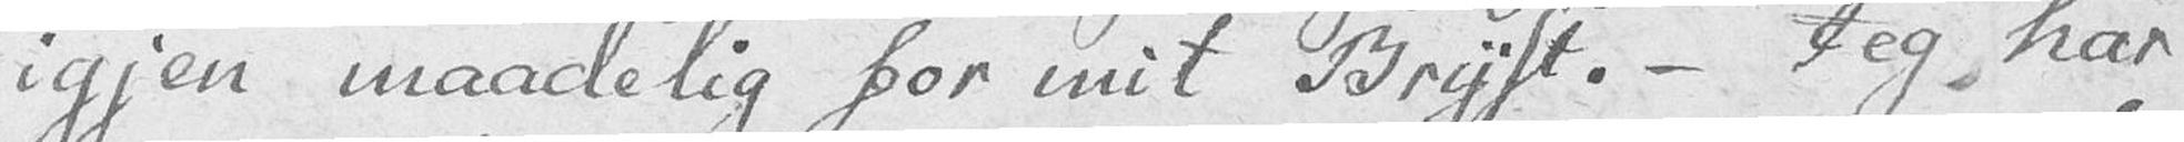igjen maadelig for mit Bryst. – Jeg har
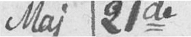Maj 21de
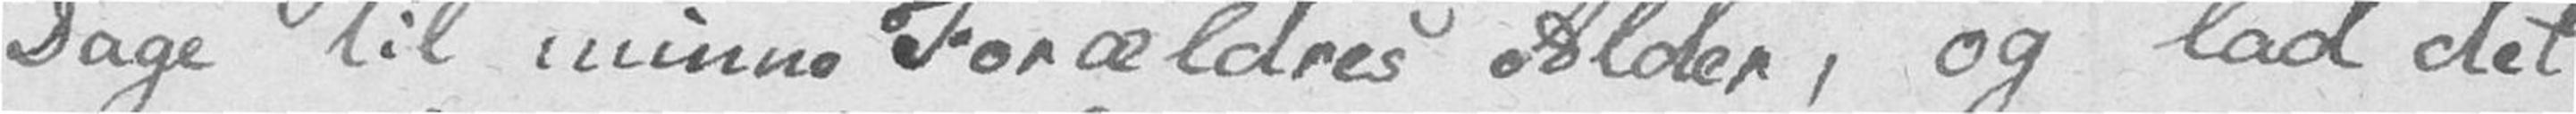Dage til minne Forældres Alder, og lad det
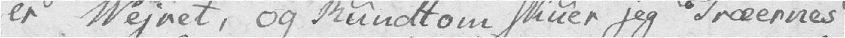er Vejret, og Rundtom skuer jeg Træernes
